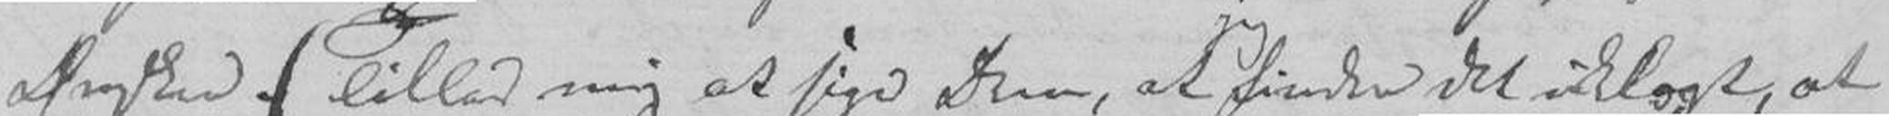Ønsker (Tillad mig at sige Den, at jeg finder det uklogt, at
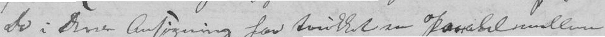De i Deres Ansøgning for trukket en paralel mellem
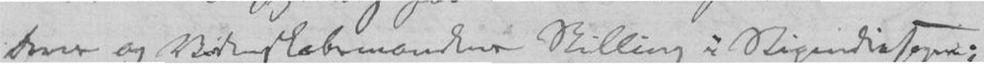Deres og Bidragskabsmanden Stilling i Stipendiesagen;
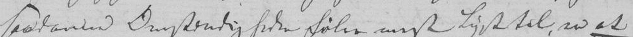saadanne Omstendigheder føler mest Lyst til, er
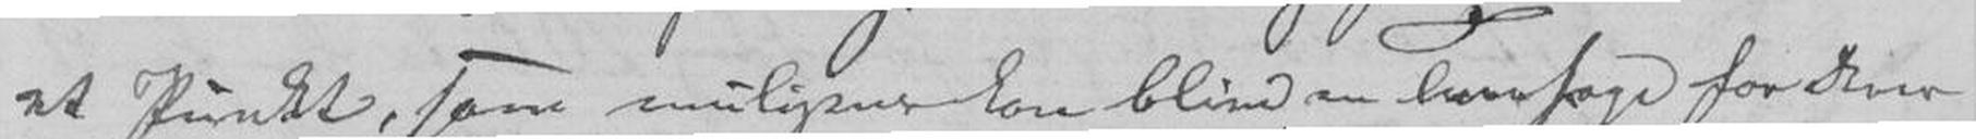et Punkt, som muligens kan blive en T...hage for disse
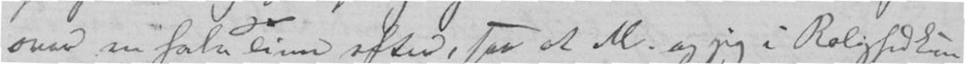over en halv Time efter, saa at M. og jeg i Rolighed kun-
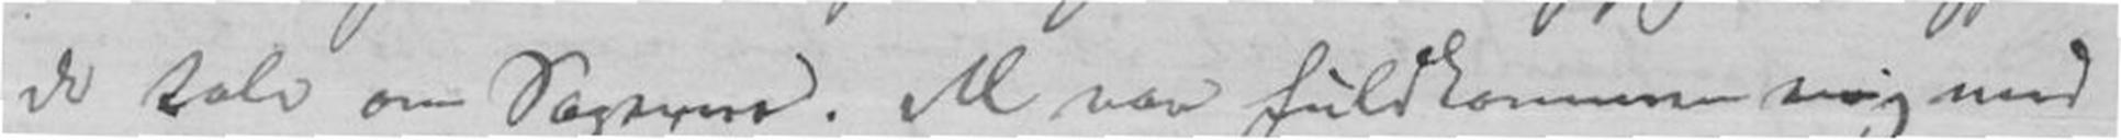de tale om Sagene. M. var fuldkommen enig med
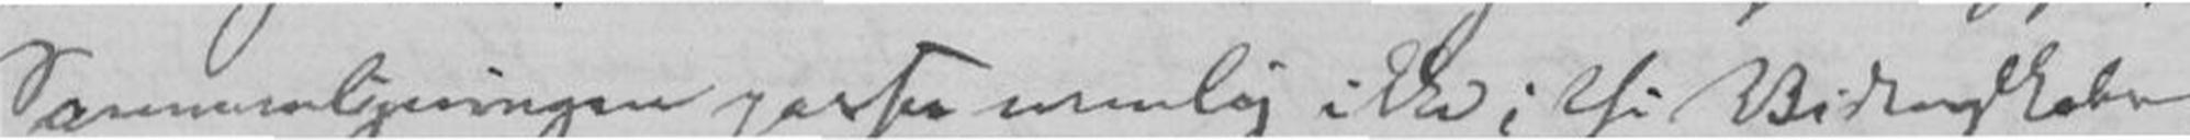Sammenligningen passer nemlig ikke; thi Bidragskaps-
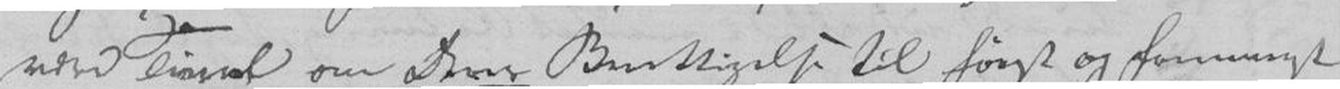være tvivl om Deres Berettigelse til først og fremst
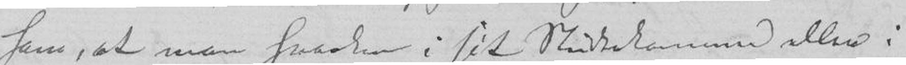ham, at man saadan i sit Studiekammer eller i
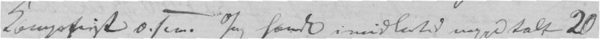Komponist o.s.v. Jeg havde imidlertid megettalt 20
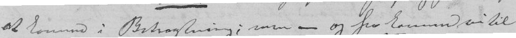at komme i Betragtning; men - og her kommer vi til
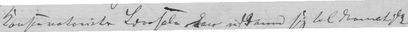Konservatoriske Love selv kan uddanne sig til dramatiske
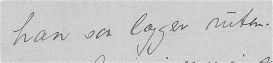han saa lægger ruten.
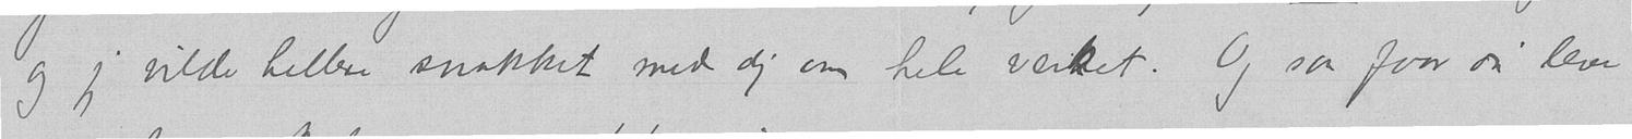og jeg vilde hellere snakket med dig om hele verket. Og saa faar du leve
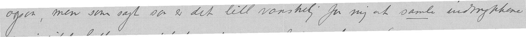ogsaa, men som sagt saa er det litt vanskelig for mig at samle indtrykkene
